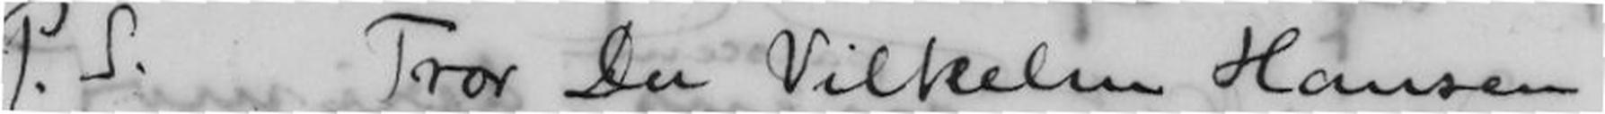P.S. Tror Du Vilhelm Hansen
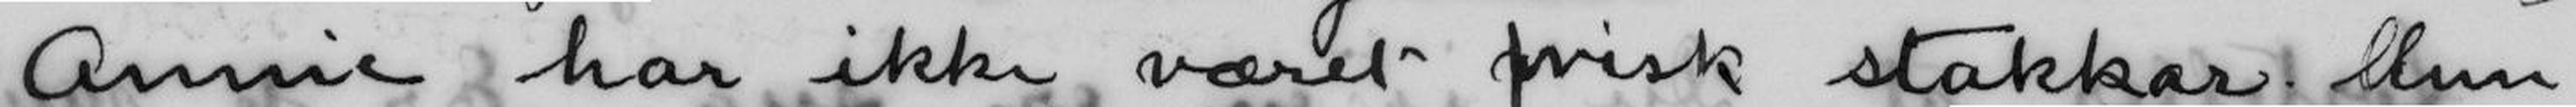Annie har ikke været frisk stakkar. Hun
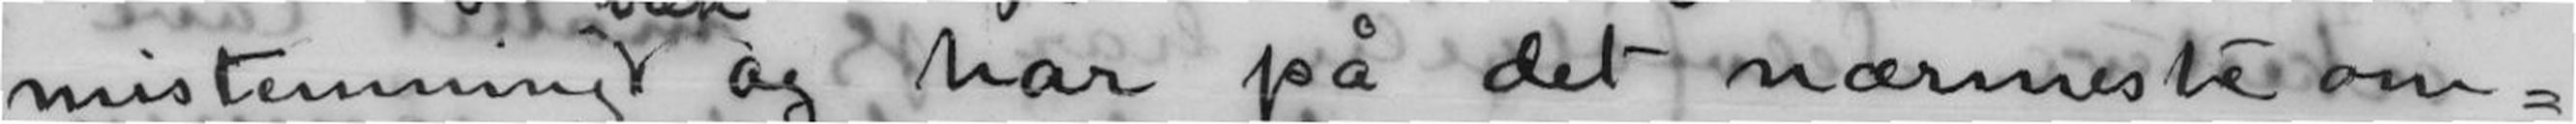mistemning og har på det nærmeste om-
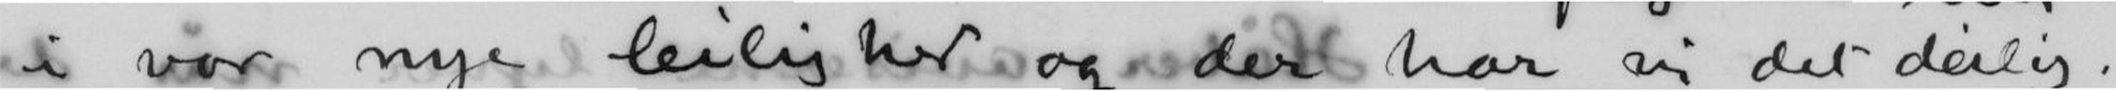i vår nye leilighed og der har vi det deilig.
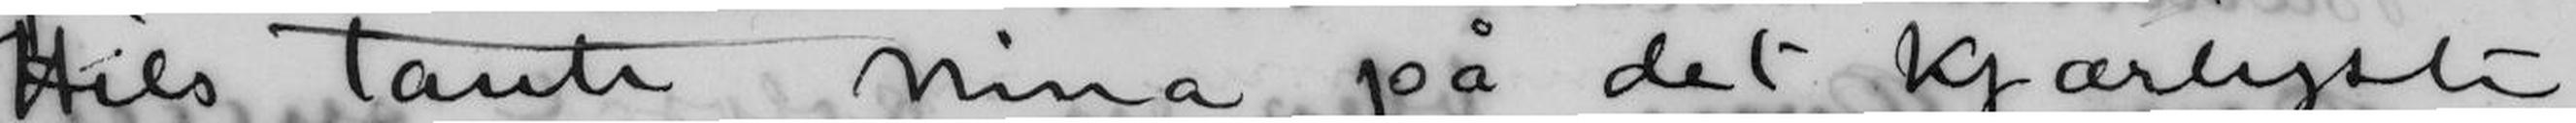Hils tante Nina på det kjærligste
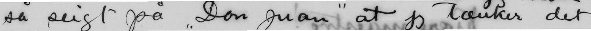så seigt på Don Juan at jeg tænker det
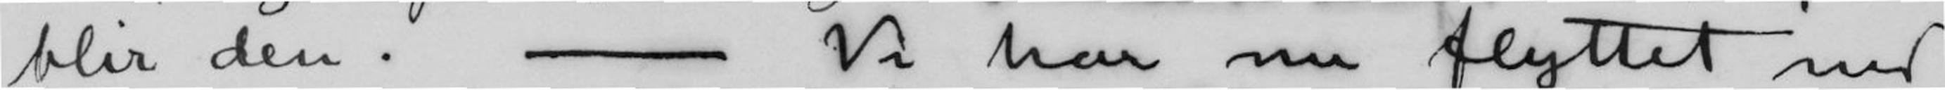blir den. - Vi har nu flyttet ind
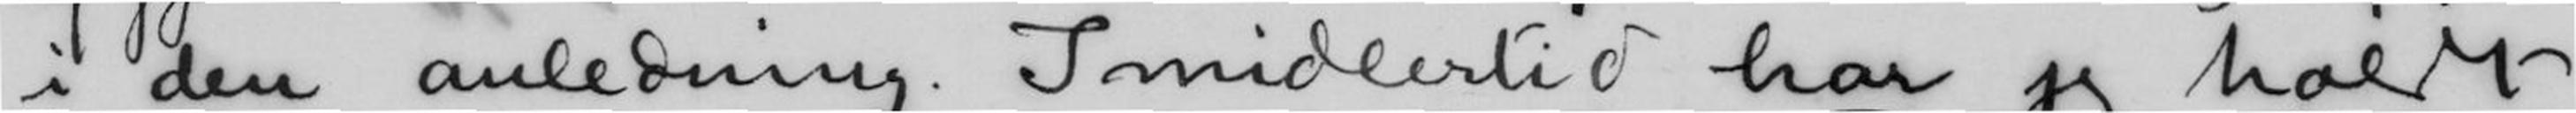i den anledning. Imidlertid har jeg holdt
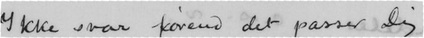Ikke svar førend det passer Dig
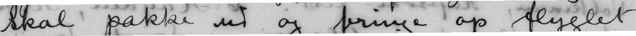skal pakke ud og bringe op flyglet
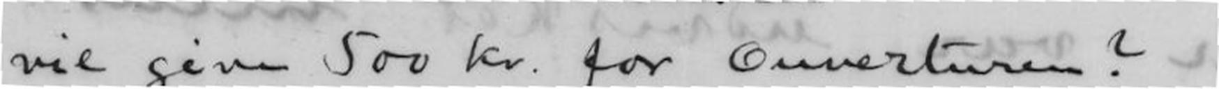vil give 500 kr. for Ouverturen?
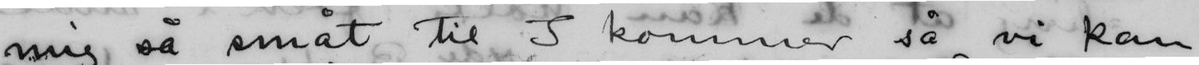mig så småt til I kommer så vi kan
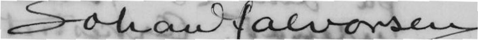Johan Halvorsen
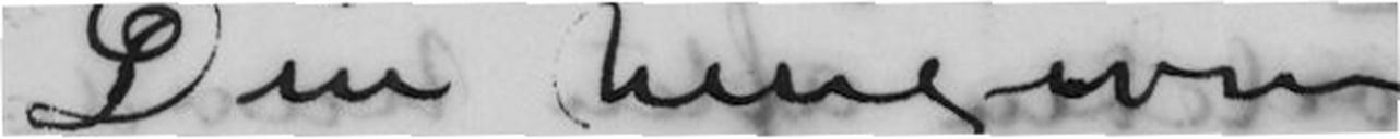Din hengivne
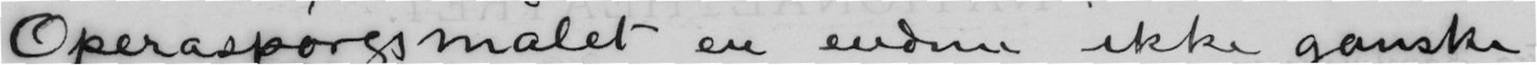Operaspørgsmålet er endnu ikke ganske
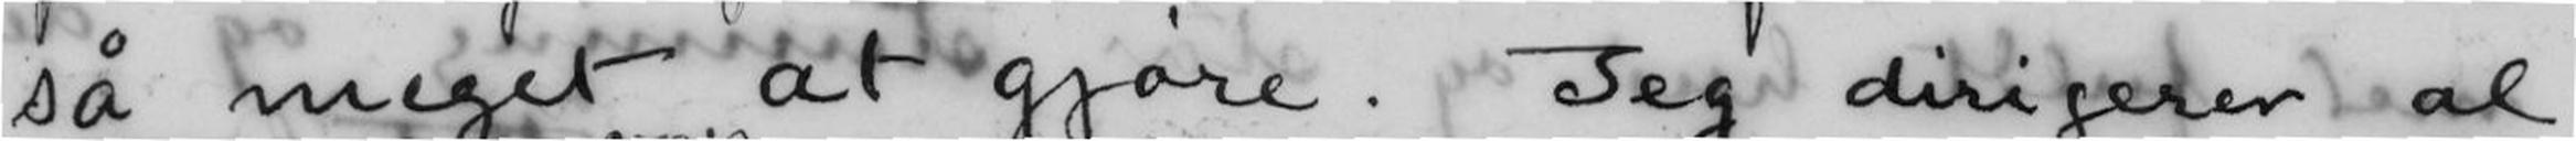så meget at gjøre. Jeg dirigerer al
